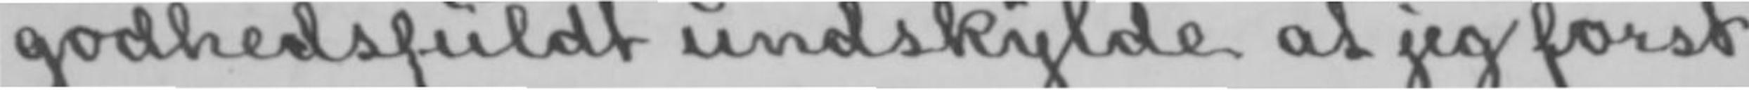godhedsfuldt undskylde at jeg først
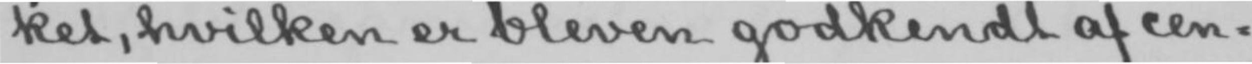ket, hvilken er bleven godkendt af cen-
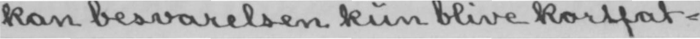kan besvarelsen kun blive kortfat-
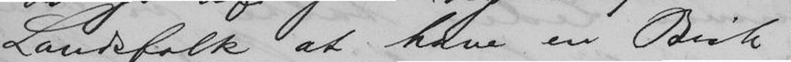Landsfolk at have en Bisk.
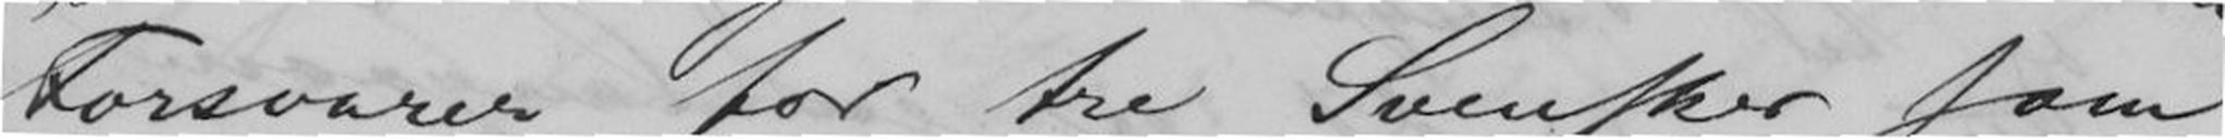Forsvarer for tre Svensker som
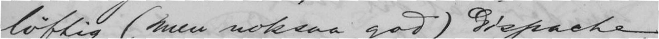løftig (men noksaa god) Dispache,
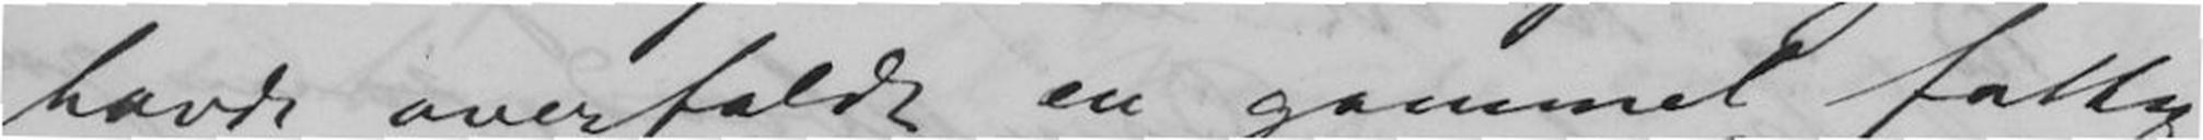havde overfaldt en gammel fattig
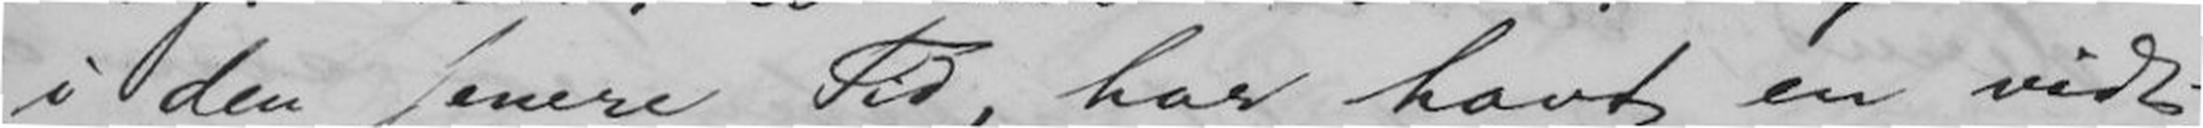i den senere Tid, har havt en vidt-
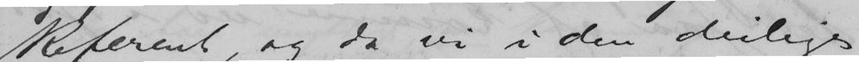Referent, og da vi i den deilige
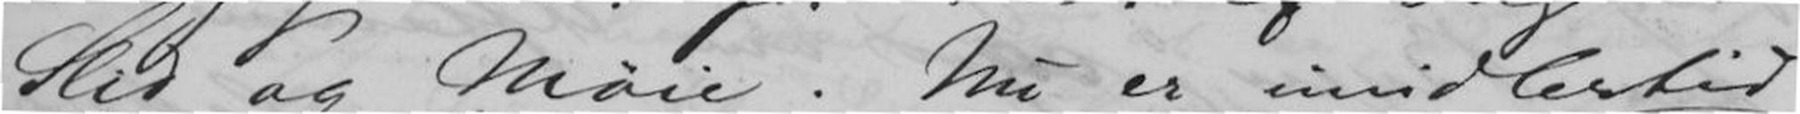Slid og Møie. Nu er imidlertid
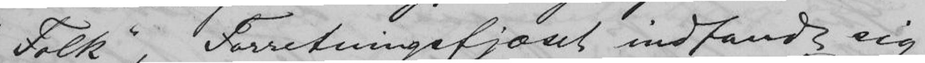"Folk", Forretningsfjæset indfandt sig
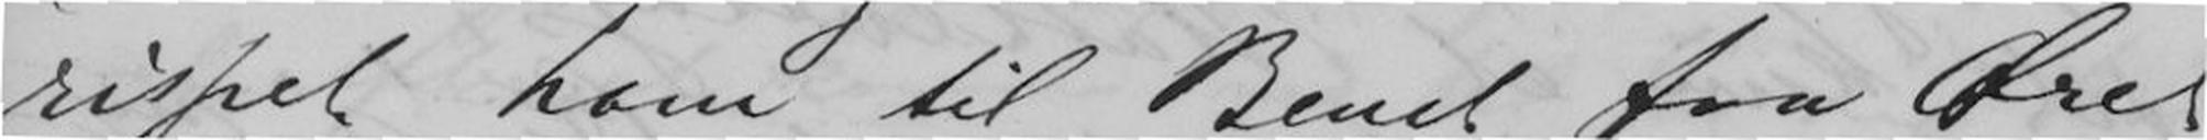rispet ham til Benet fra Øret
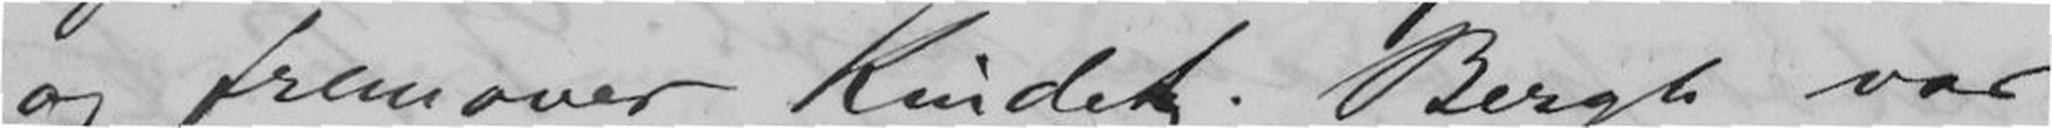og fremover Kindet. Bergh var
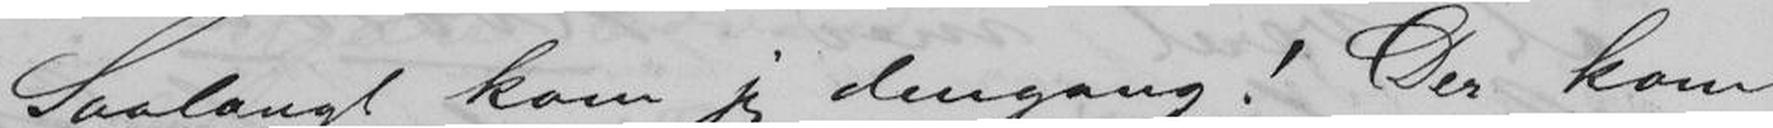Saalangt kom jeg dengang! Der kom
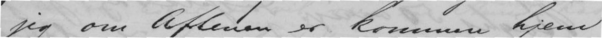jeg om Aftenen er kommen hjem
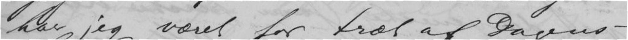har jeg været for træt af Dagens -
